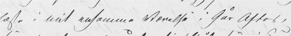læste i mit ensomme Værelse i Går Aftes,
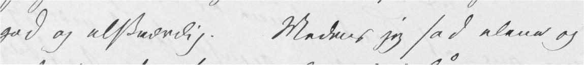god og elskværdig. Medens jeg sad alene og
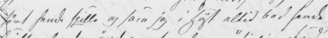hørt hende spille og som jeg i Høst altid bad hende
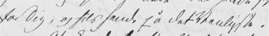for Dig, og hils hende på det Venligste.
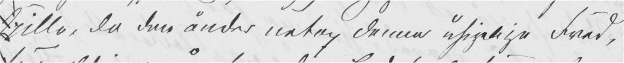spille, da den ånder netop denne usigelige Fred,
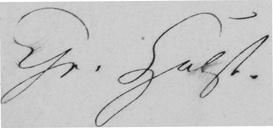Chr. Holst.
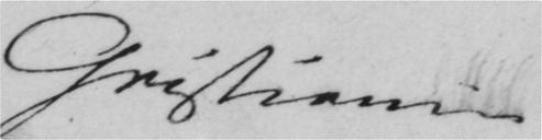Christiania
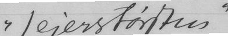"sejerstørsten")
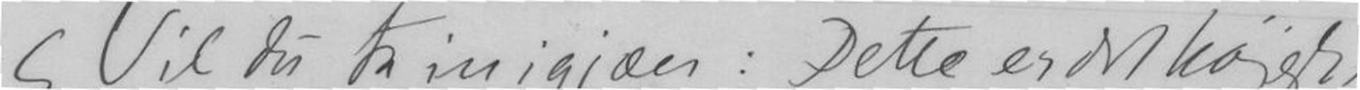Vil du ta in igjen: Dette er det højeste
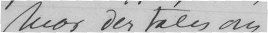hvor der tales om
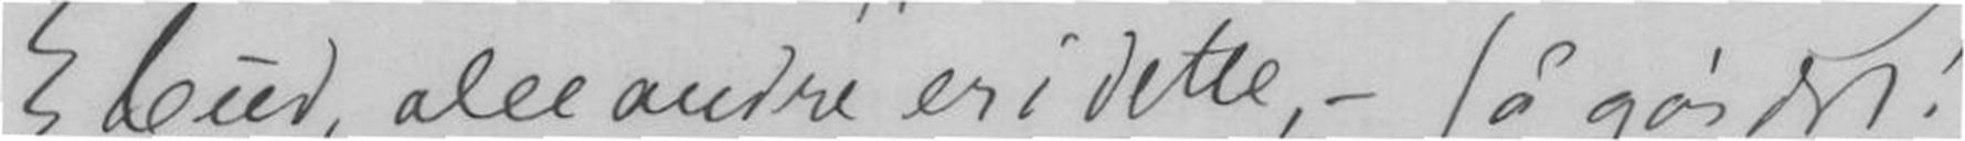bud, alle andre er i dette, - så gør det!
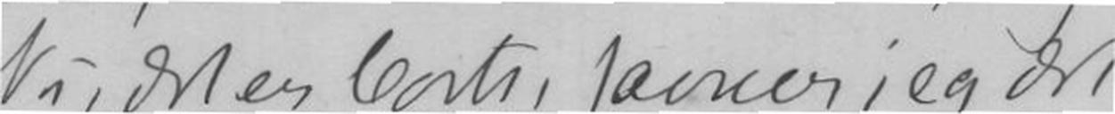Nu, det er borte, savner jeg det.
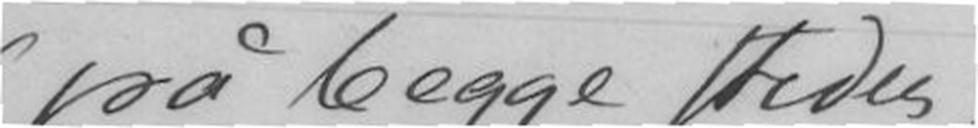(på begge steder,
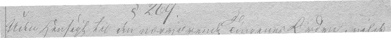Uden Hensigt til den nærværende Tingenes Orden, vilde
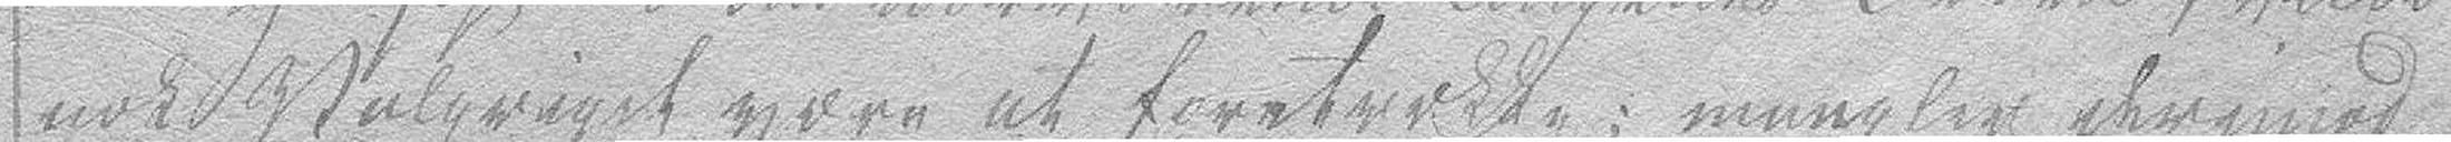nok Valgriget være at foretrække; mangler derimod
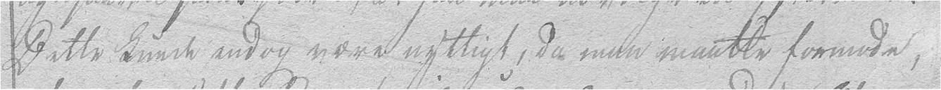Dette kunde endog være nyttigt, da man maatte formode,
